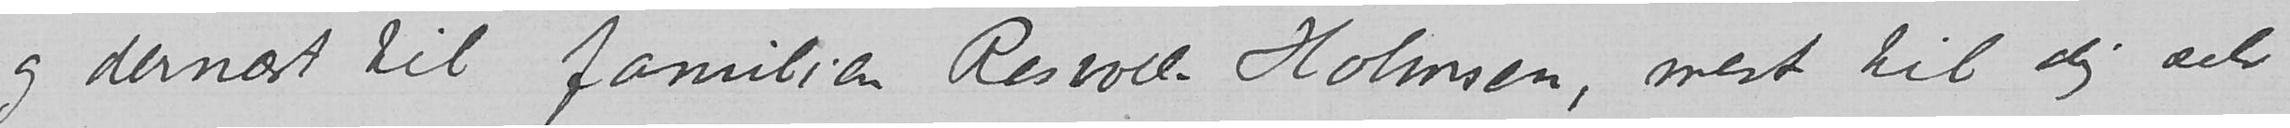og dernæst til familien Resvoll Holmsen, mest til dig selv
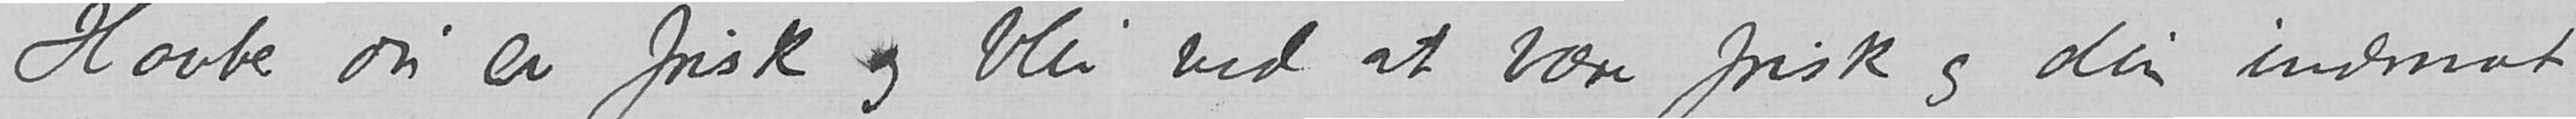Haaber du er frisk og blir ved at være frisk og din indmat
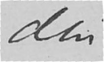din
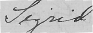Sigrid
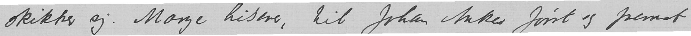skikker sig. Mange hilsener, til Johan Anker først og fremst
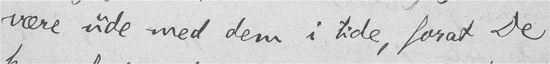være ude med dem i tide, forat De
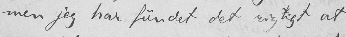men jeg har fundet det rigtigt at
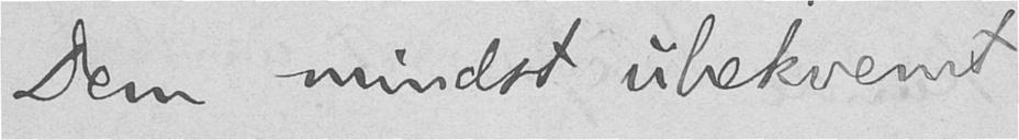Dem mindst ubekvemt.
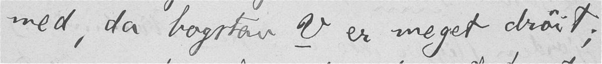med, da bogstav V er meget drøit;
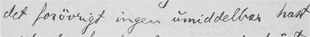det forøvrigt ingen umiddelbar hast
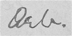Ærb.
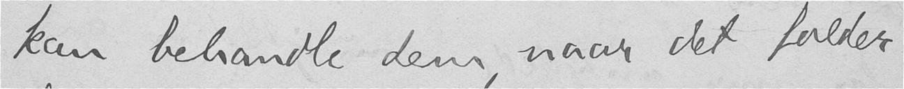kan behandle dem, naar det falder
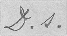D. s.
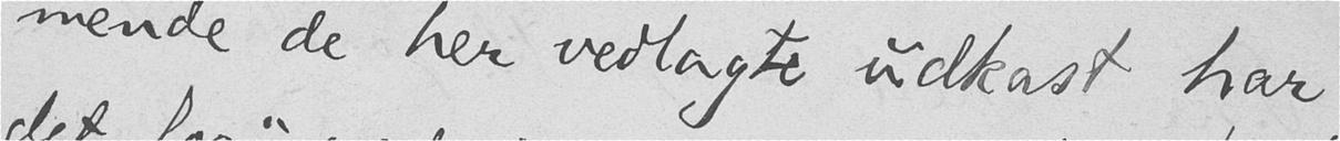mende de her vedlagte udkast har
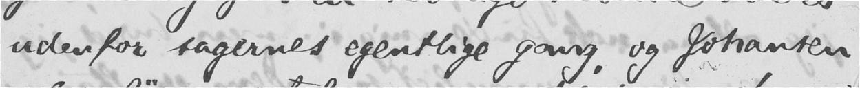udenfor sagernes egentlige gang, og Johansen
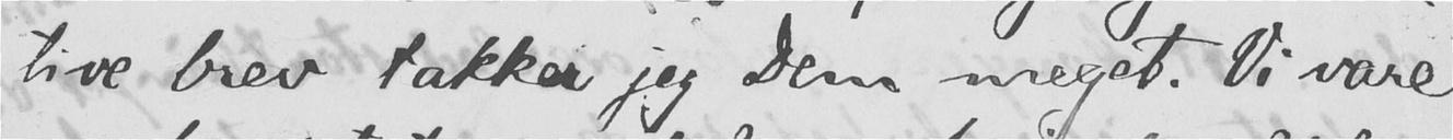tive brev takker jeg Dem meget. Vi vare
